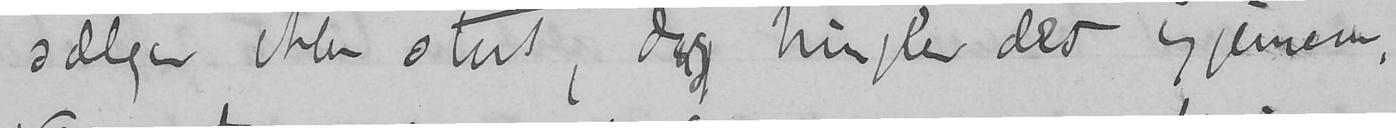sælger ikke stort, dog hungler det igjennem.
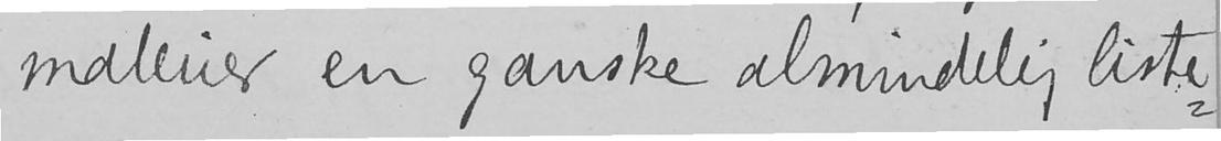malerier en ganske almindelig liste-
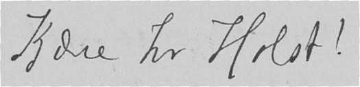Kjære hr Holst!
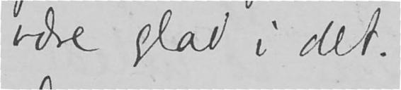være glad i det.
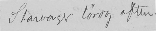Stavanger lørdag aften.
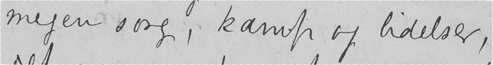megen sorg, kamp og lidelser,
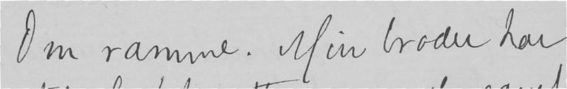Om ramme. Min broder har
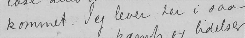kommet. Jeg lever her i saa
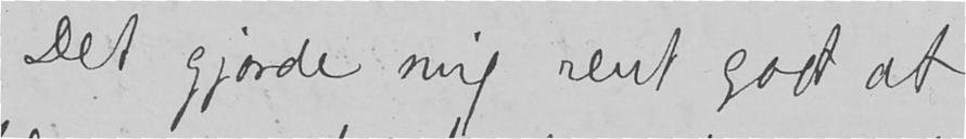Det gjorde mig rent godt at
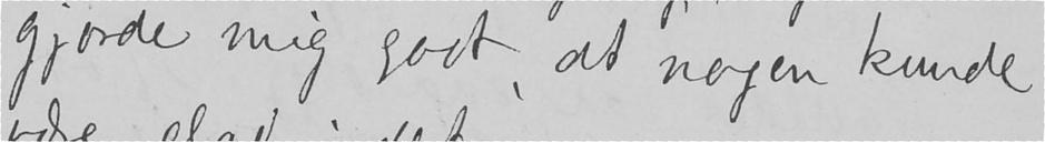gjorde mig godt, at nogen kunde
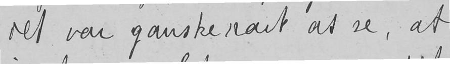det var ganske rart at se, at
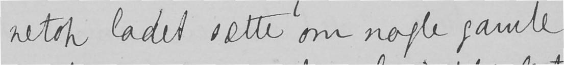netop ladet sætte om nogle gamle
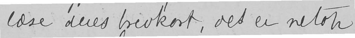læse deres brevkort, det er netop
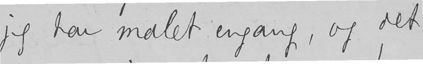jeg har malet engang, og det
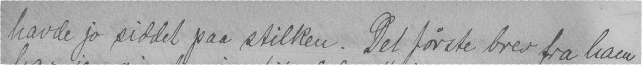havde jo siddet paa stilken. Det første brev fra ham
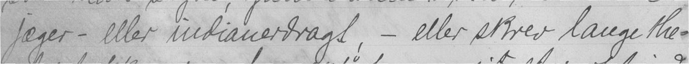jæger - eller indianerdragt, - eller skrev lange the-
